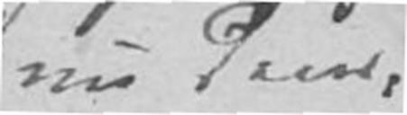nu dens
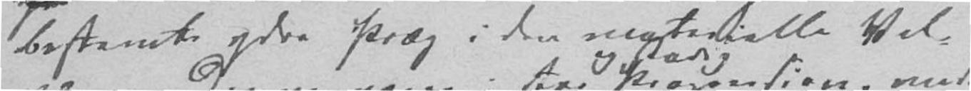bestemt ydre Præg i den materielle Vel-
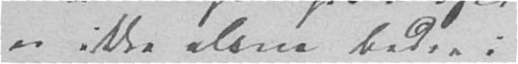er ikke alene bedre i
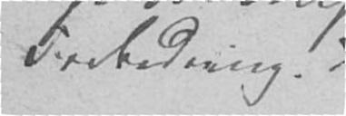Forbedning.
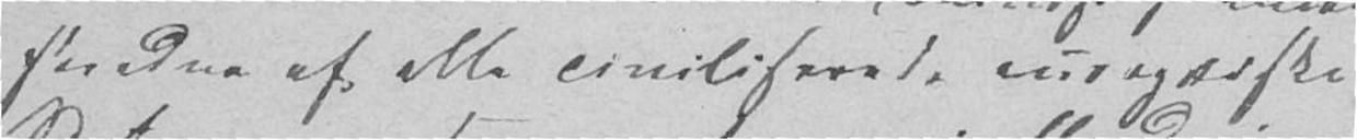skredne af alle civiliserede ungarske
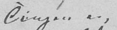Tingen er,
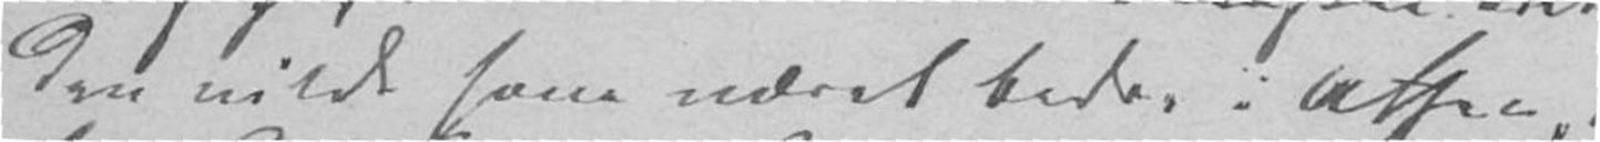den vilde have været bedre i Athen
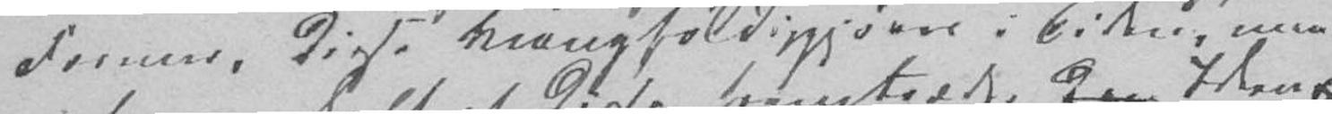Former, disse mangfoldiggjøres i Tiden, men
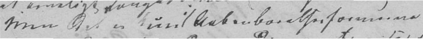Men det er kun i Aabenbarelsesformerne
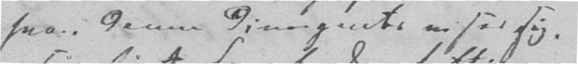hvor denne Divergents viser sig.
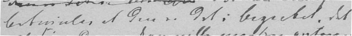betvivles at den er det i Begrebet, det
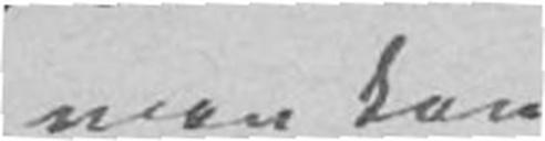man kan
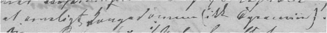et arveligt Kongedømme (ikke Tyrannie).
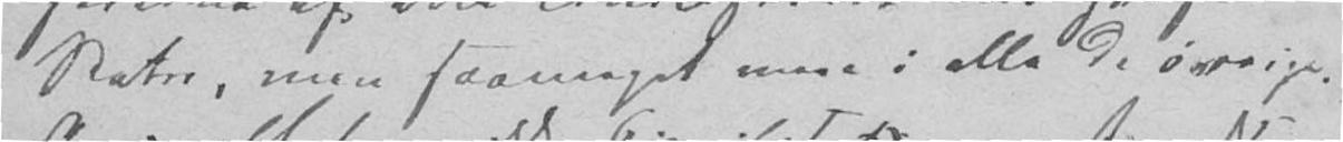Stater, men saameget mere i alle de øvrige.
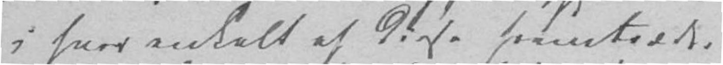i hver enkelt af disse fremtræder
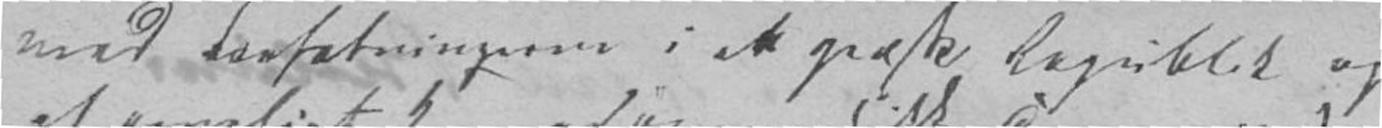med Forfatningerne i en græsk Republik og
